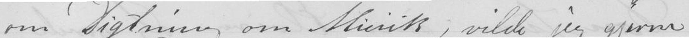om Digtning om Musik, vilde jeg gjerne
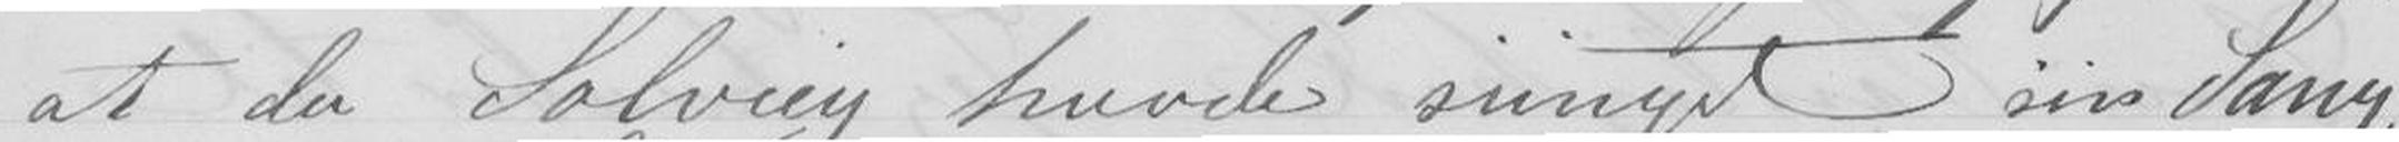at da Solveig havde sunget sin Sang,
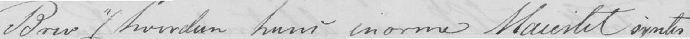Brev "(hvordan hans enorme Maiestet syntes
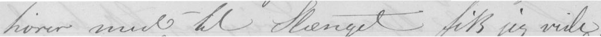hører med til Slænget fik jeg vide
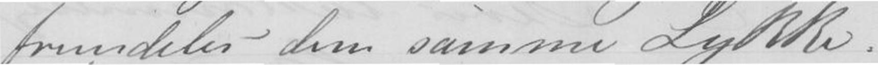fremdeles den samme Lykke.
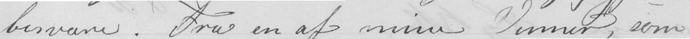bevare. Fra en af mine Venner, som
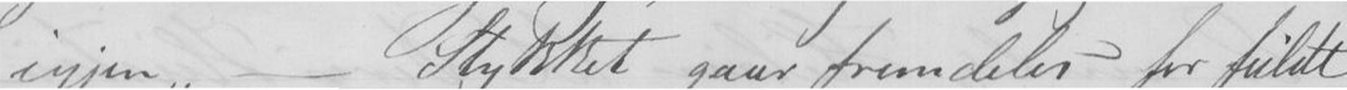igjen _ Stykket gaar fremdeles for fuldt
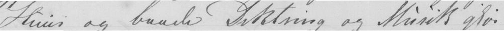Huus og baade Diktning og Musik gjør
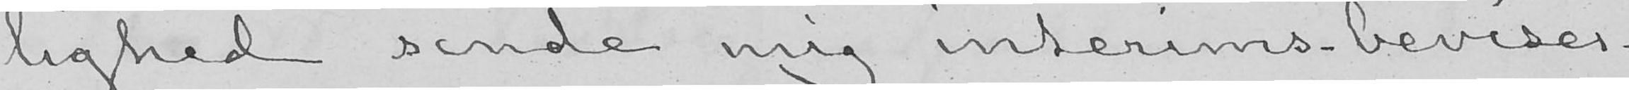lighed sende mig interims-beviser-
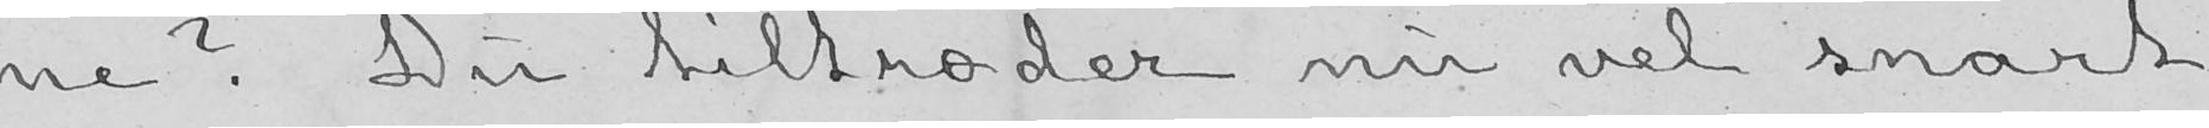ne? Du tiltræder nu vel snart
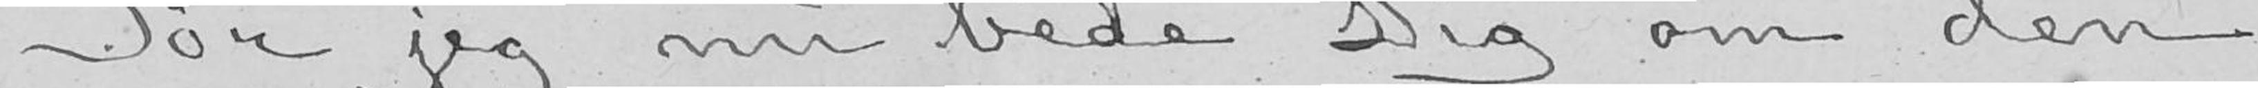Tør jeg nu bede Dig om den
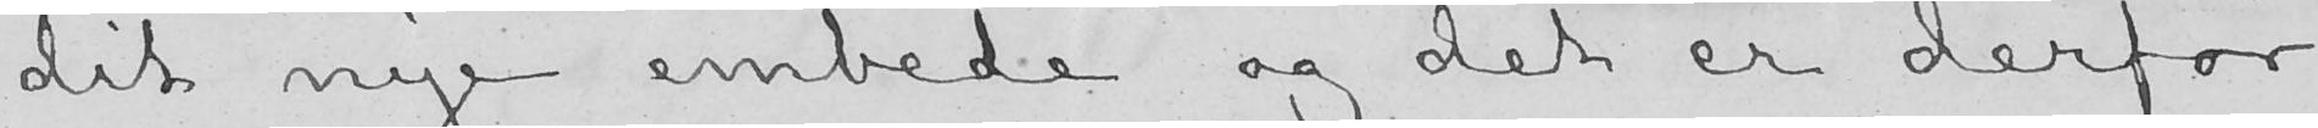dit nye embede og det er derfor
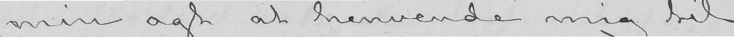min agt at henvende mig til
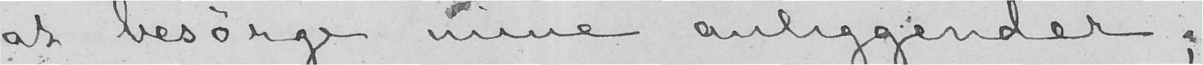at besørge mine anliggender;
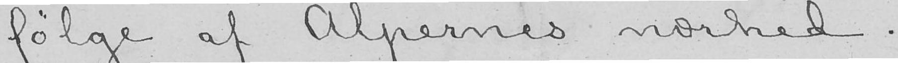følge af Alpernes nærhed.
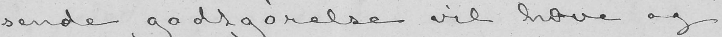sende godtgørelse vil hæve og
